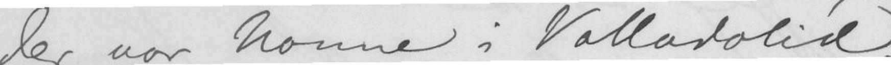der var nonne i Valladolid.
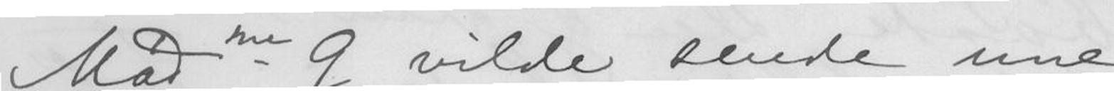Mádme G vilde sende une
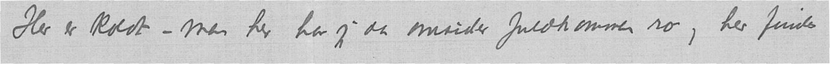Her er koldt – men her har jeg da omsider fuldkommen ro, og her findes
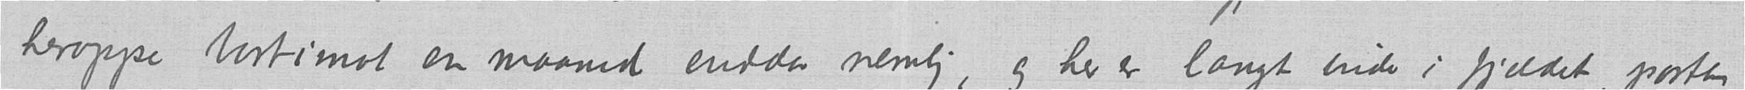heroppe bortimot en maaned endda nemlig, og her er langt inde i fjeldet, posten
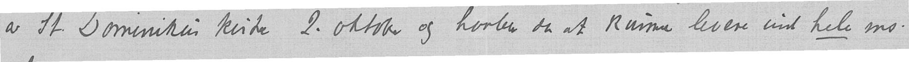av St. Dominikus kirke 2. oktober og haaber da at kunne levere ind hele ms.
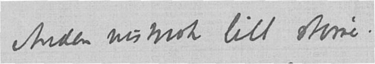Anden vistnok litt større.
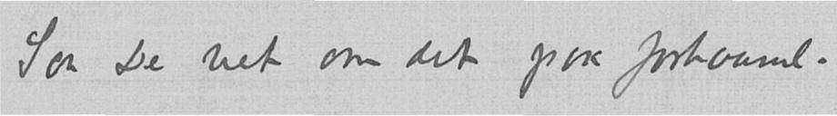Saa De vet om det paa forhaand.
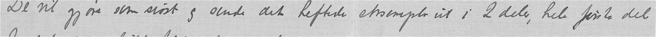De vil gjøre som sisst og sende det heftede eksemplar ut i 2 deler, hele første del
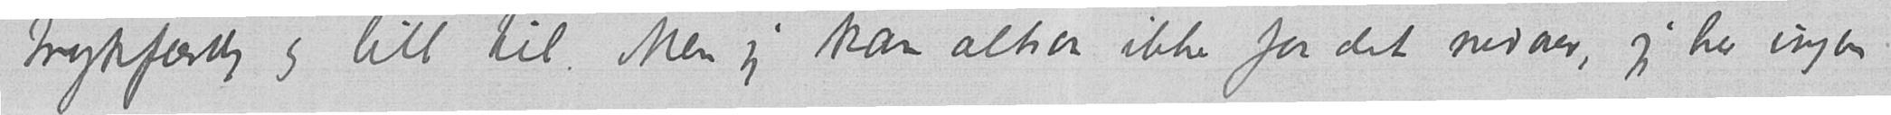trykfærdig og litt til. Men jeg kan altsaa ikke faa det nedover, jeg har ingen
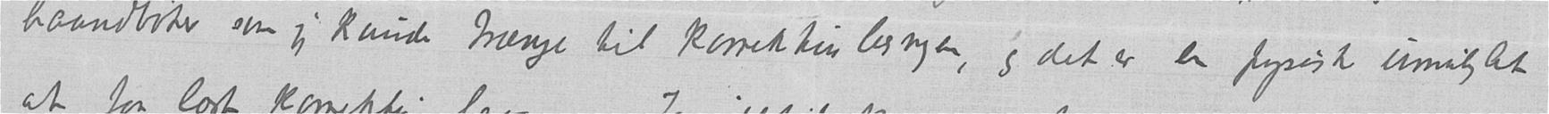haandbøker som jeg kunde trænge til korrekturlæsningen, og det er en fysisk umulighet
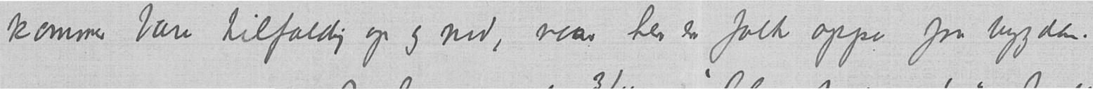kommer bare tilfældig op og ned, naar her er folk oppe fra bygden.
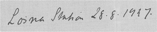Losna Station 28.8.1927.
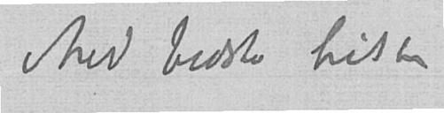Med bedste hilsen
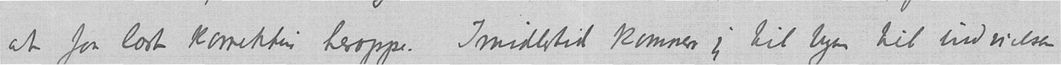at faa læst korrektur heroppe. Imidlertid kommer jeg til byen til indvielsen
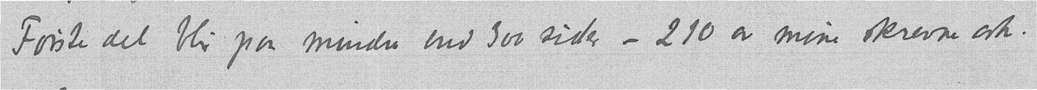Første del blir paa mindre end 300 sider – 210 av mine skrevne ark.
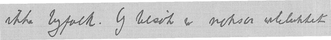ikke byfolk. Og besøk er noksaa urtelukket
